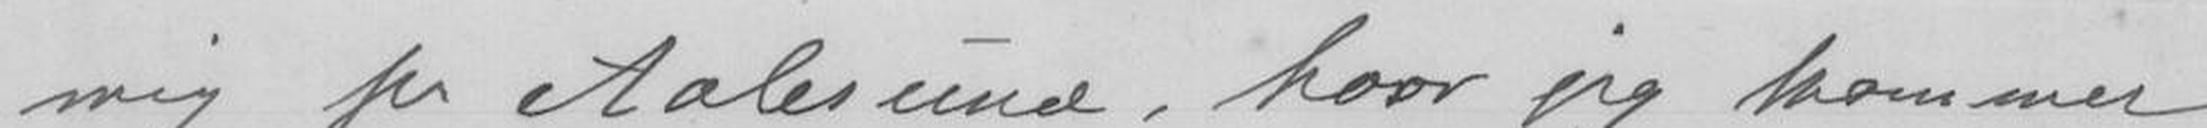mig pr Aalesund, hvor jeg kommer
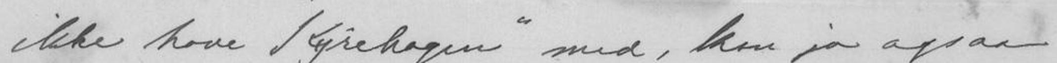ikke have "Kyrehagen" med, kan jo ogsaa
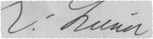E. Lund
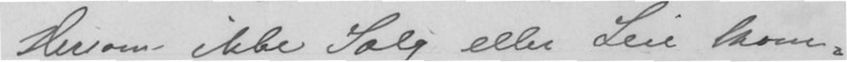Herom ikke Salg eller Leie kom-
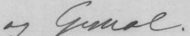og Gemal
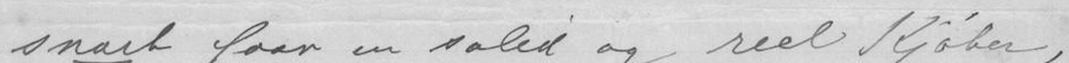snart faar en solid og reel Kjøber,
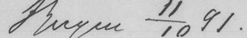Bergen 11/10 91.
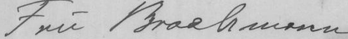Fru Brochmann
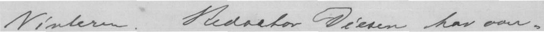Vinteren. Redaktør Diesen har over-
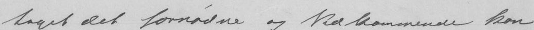taget det fornødne og Vedkommende kan
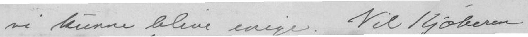vi kunne blive enige. Vil Kjøberen
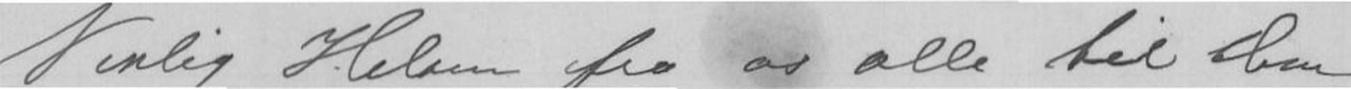Venlig Hilsen fra os alle til Dem
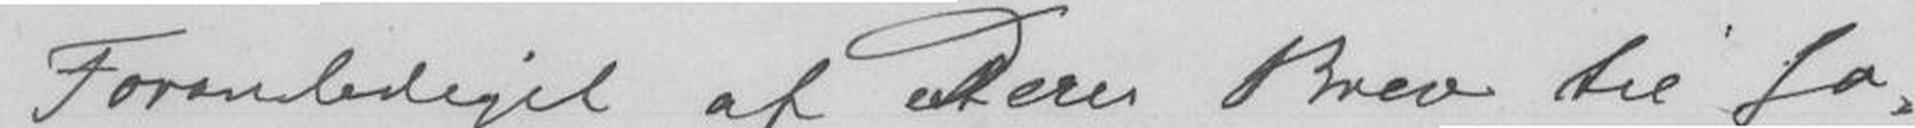Foranlediget af Deres Brev til Jo-
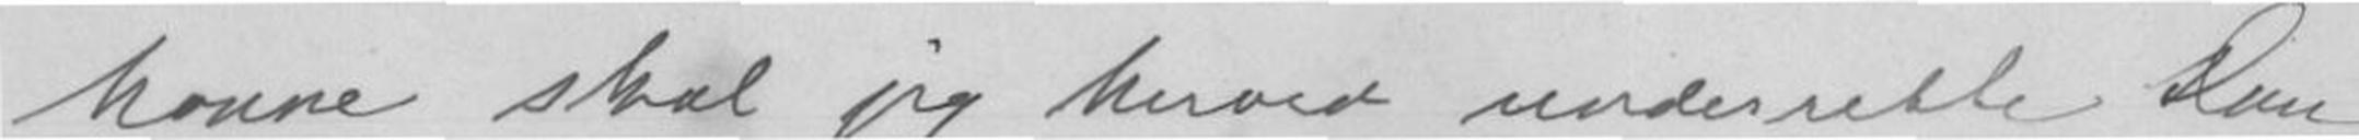hanne skal jeg herved underette Dem
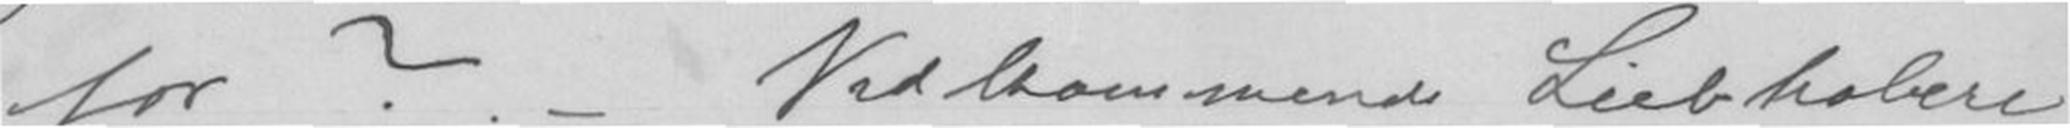for?. - Vedkommende Liebholbere
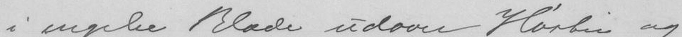i engelse Blade udover Høsten og
